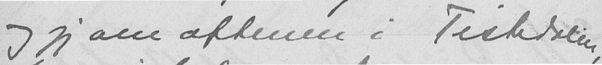og jeg om aftenen i Tistedalen,
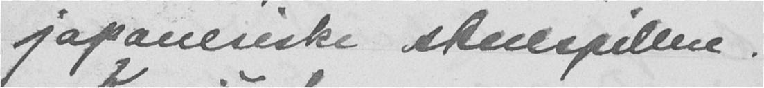japanesiske skuespillere.
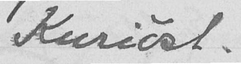Kuriøst.
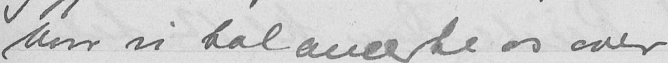hvor vi balancerte os over
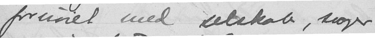fornøiet med selskab, svoger
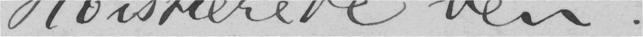Høistærede ven!
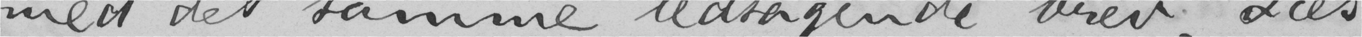med det samme ledsagende brev. Læs-
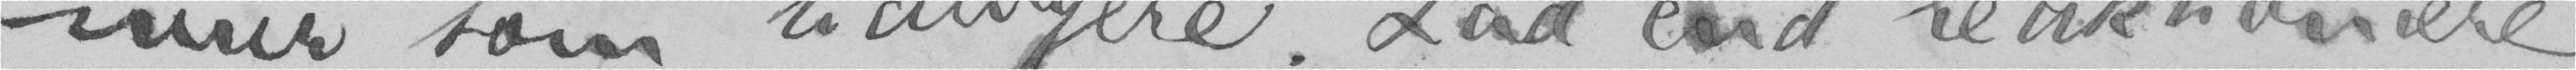mur som tidligere. Lad end reaktionære
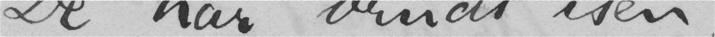De har brudt isen
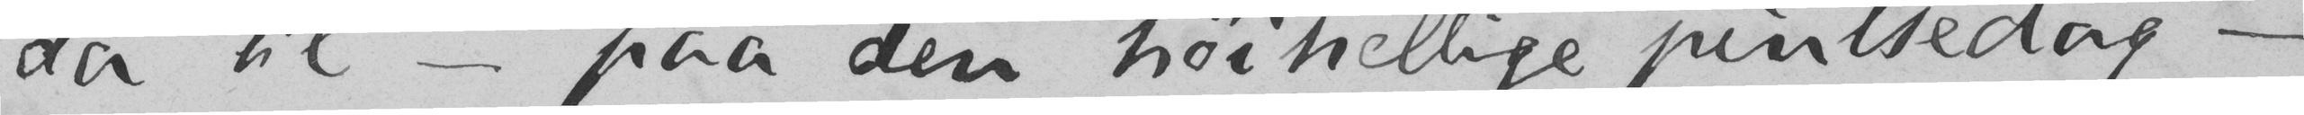da til - paa den høihellige pintsedag -
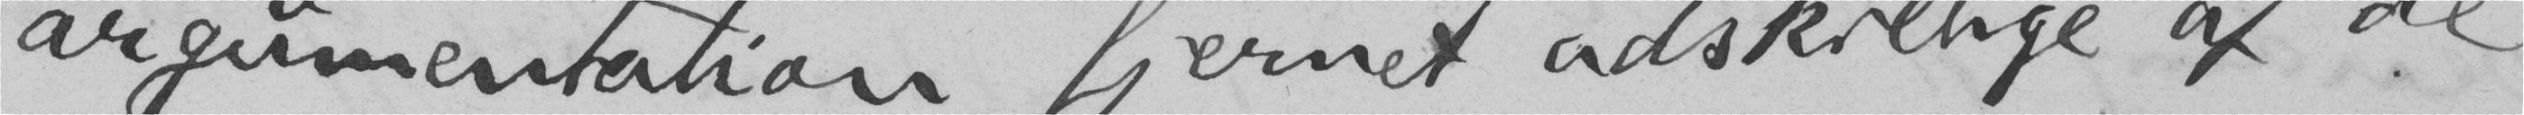argumentation fjernet adskillige af de
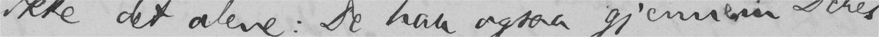ikke det alene: De har ogsaa gjennem deres
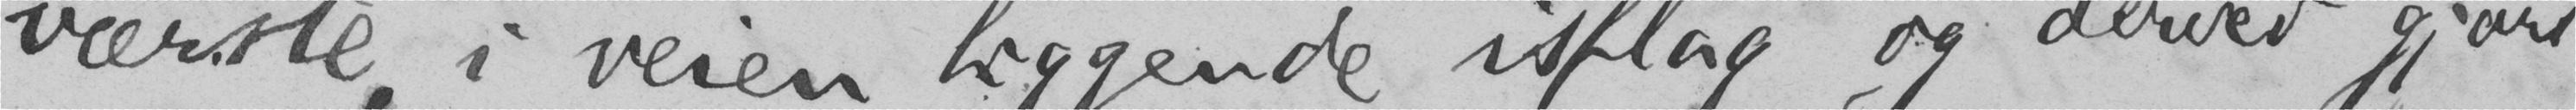værste, i veien liggende isflag og derved gjort
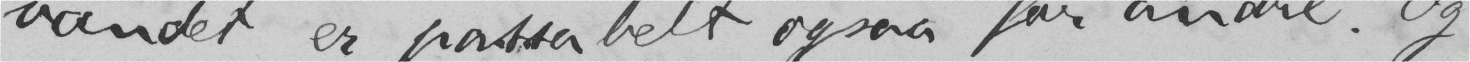vandet er passabelt ogsaa for andre. Og
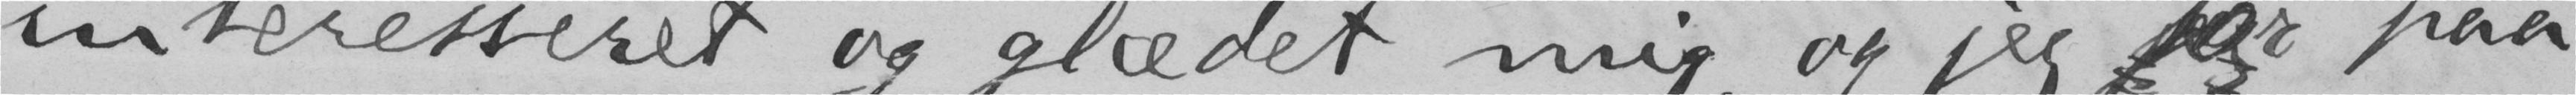interesseret og glædet mig, og jeg tør paa
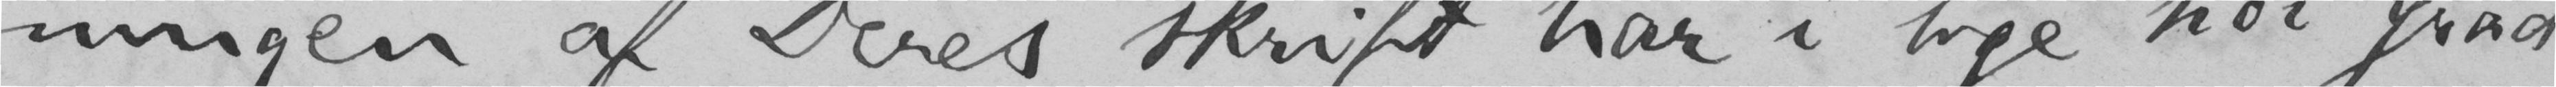ningen af Deres skrift har i lige høi grad
